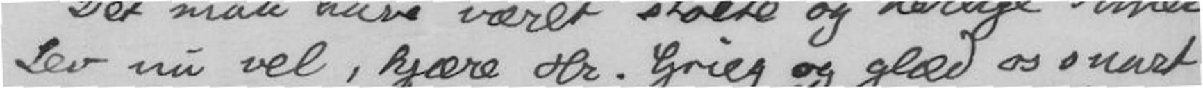Lev nu vel, kjære Hr. Grieg og glæd os snart
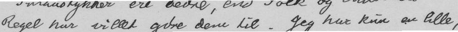Regel har villet gøre dem til. Jeg har kun en lille,
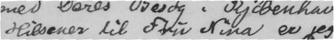Hilsener til Fru Nina er jeg
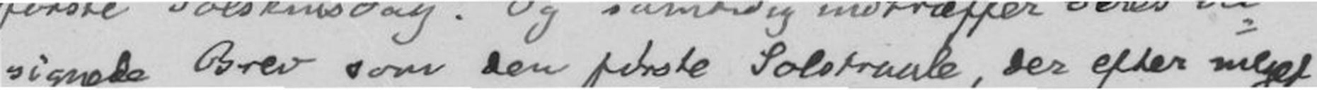signede Brev som den første Solstraale, der efter meget
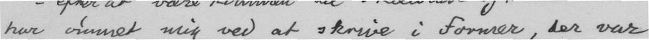har vunnet mig ved at skrive i Former, der var
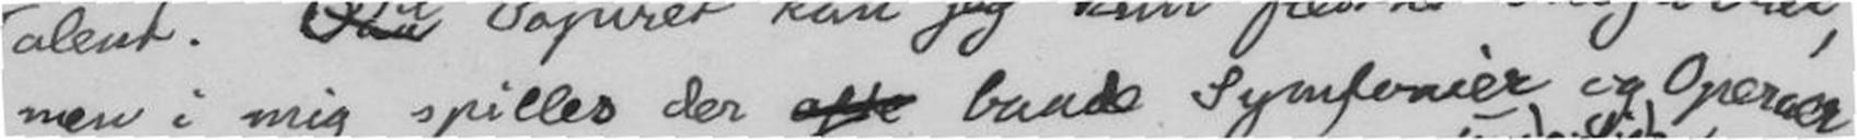men i mig spilles der ofte baade Symfonier og Operaer
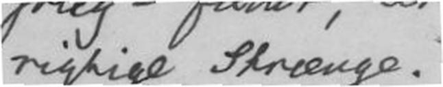rigtige Strænge.
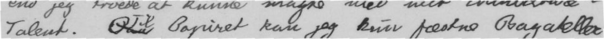Talent. Paa Til Papiret kan jeg kun fæstne Bagateller,
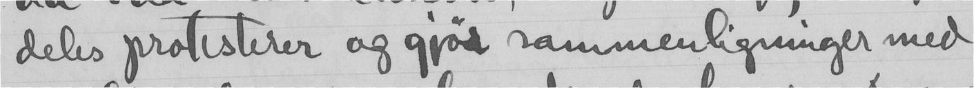deles protesterer og gjør sammenligninger med
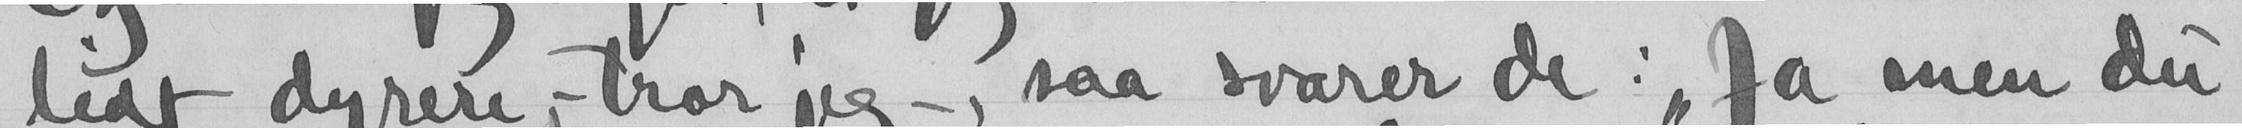lidt dyrere, - tror jeg -, saa svarer de "Ja men du
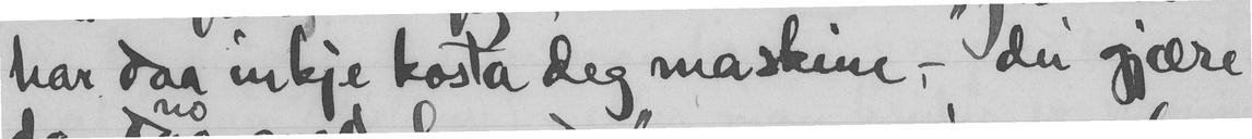har daa inkje kosta deg maskine, - der gære
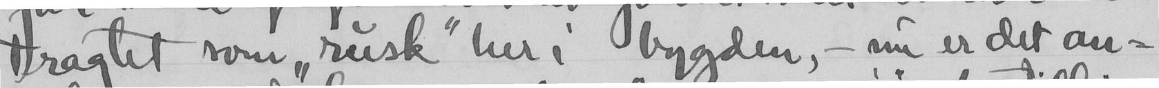tragtet som "rusk" her i bygden, - nu er det an-
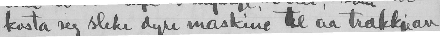kosta seg sleke dyre maskine te aa trækkjeav
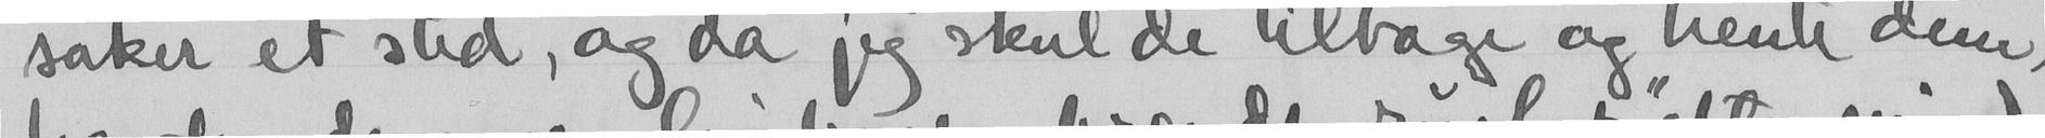saker et sted, og da jeg skulde tilbage og hente dem,
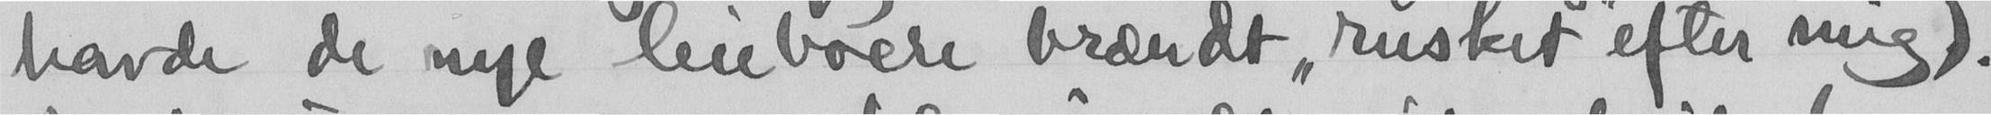havde de nye leieboere brændt "rusket" efter mig).
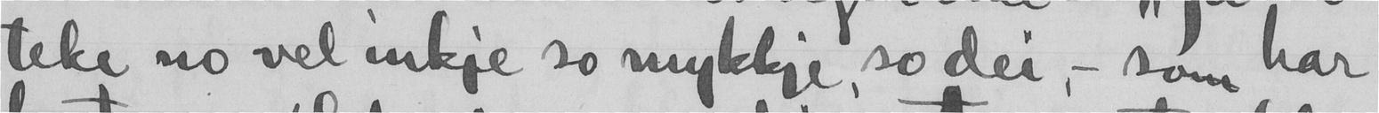teke no vel inkje so mykkje, so dei, - som har
